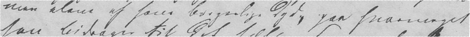men alene af hans borgerlige Dyd, paa hvormeget
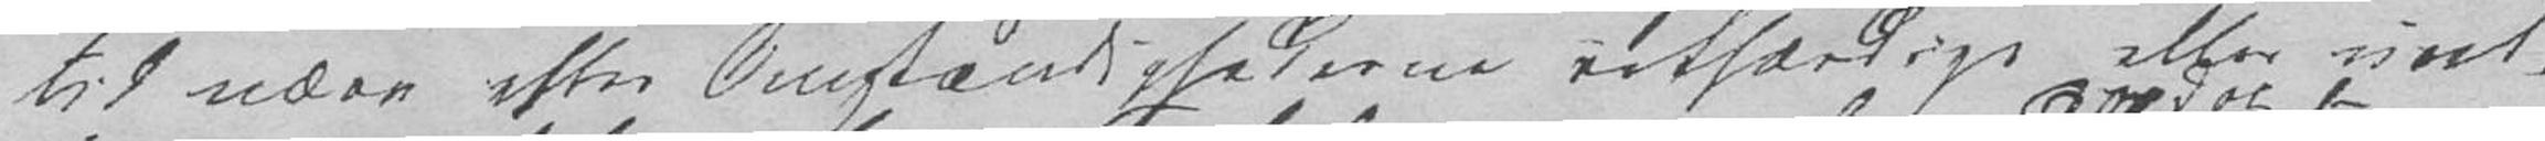tid være efter Omstændighederne retfærdige eller uret-
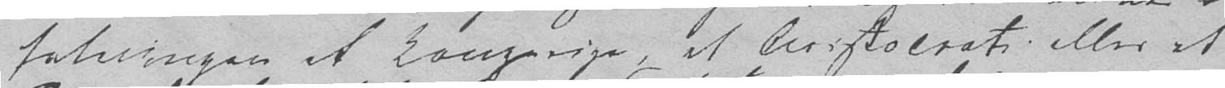fatningen et Kongerige, et Aristocrati eller et
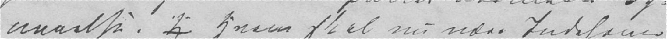naaelse. H Hvem skal nu være Indehaver
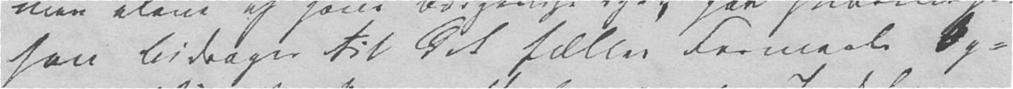han bidrager til det fælles Formaals Op-
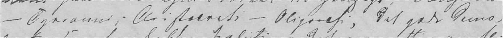– Tyranni, Aristocrati – Oligarchi, det gode Demo-
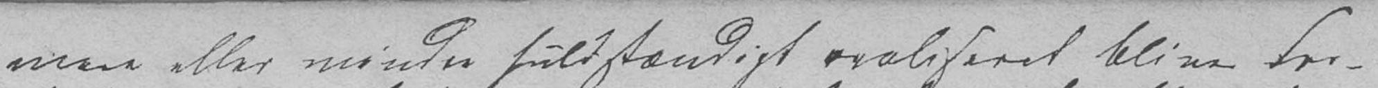mere eller mindre fuldstændigt urealiseret bliver For-
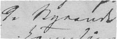de Styrende
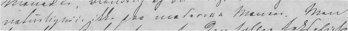naturligviis ikke paa moderne Maneer. Men
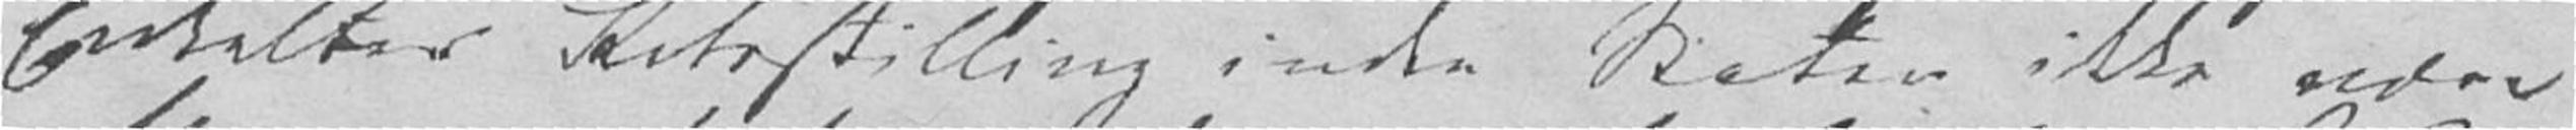Enkeltes Retsstilling inden Staten ikke være
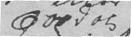gresk?
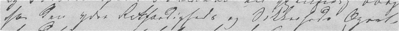for den ydre Retfærdigheds og Sikkerheds Opret-
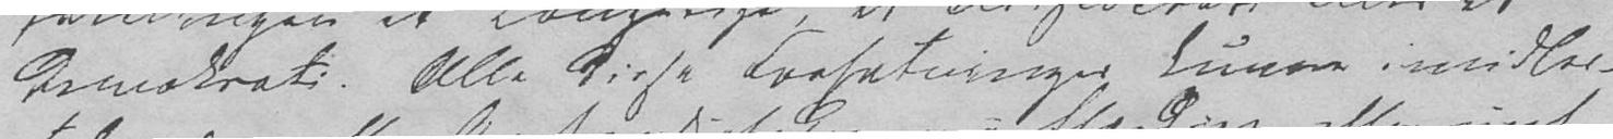Demokrati. Alle disse Forfatninger kunne imidler-
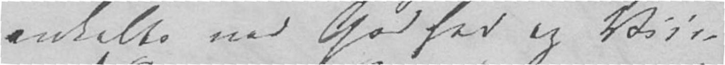enkelte ved Godhed og Viis-
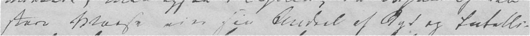store Masse vier sin Andeel af Dyd og Intelli-
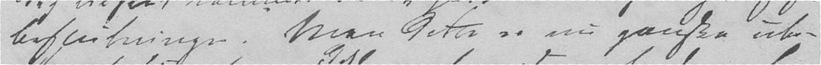Beslutninger. Men dette er nu ganske ube-
